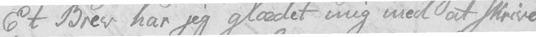Et Brev har jeg glædet mig med at skrive
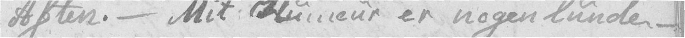Aften. – Mit Humeur er nogenlunde. –
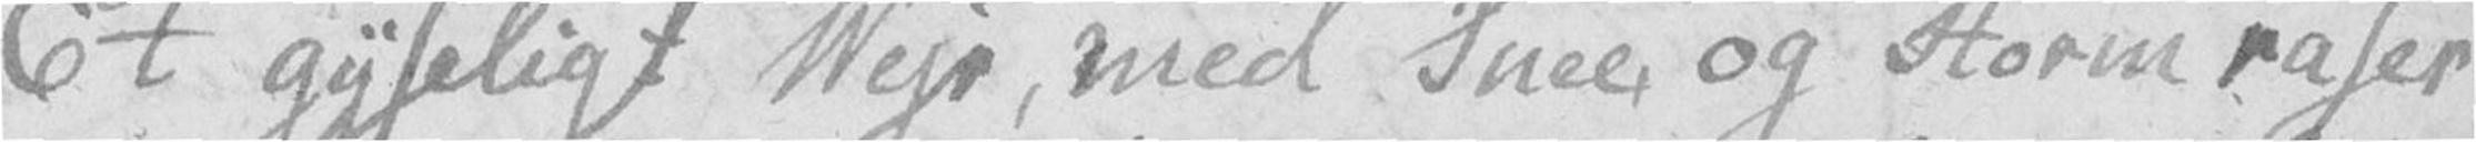Et gyseligt Vejr, med Snee, og Storm raser
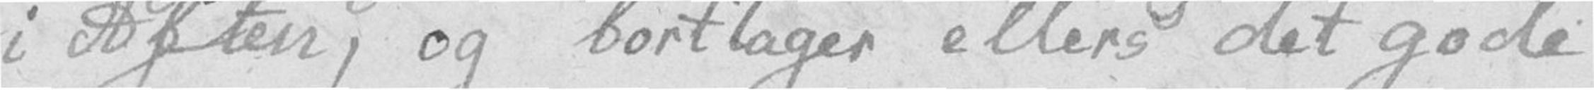i Aften, og borttager ellers det gode
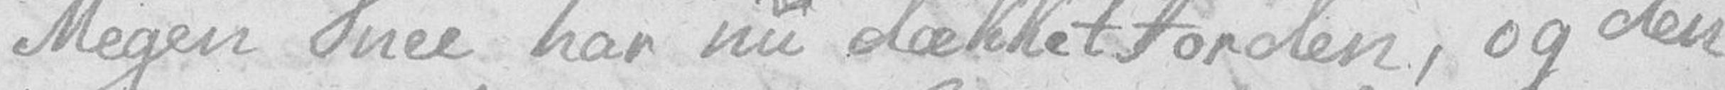Megen Snee har nu dækket Jorden, og den
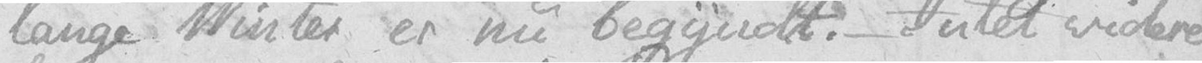lange Vinter er nu begyndt. – Intet videre
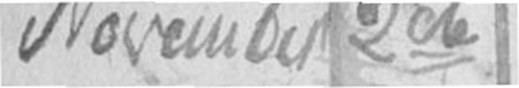November 2de
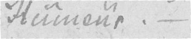Humeur. –
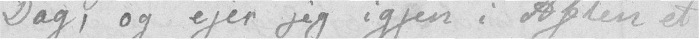Dag, og ejer jeg igjen i Aften et
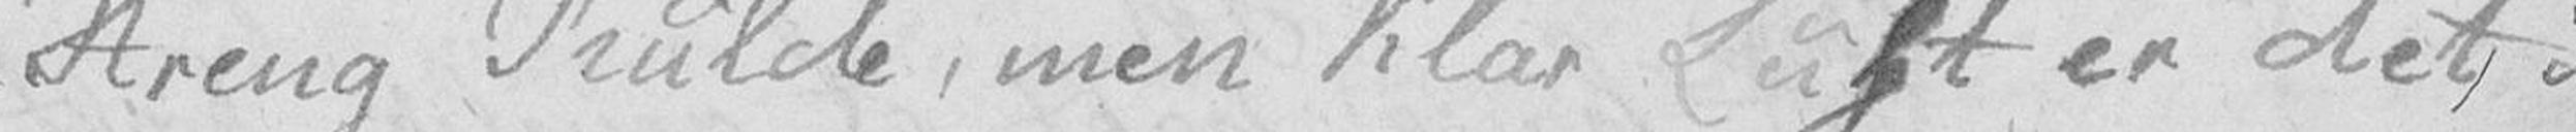Streng Kulde, men klar Luft er det i
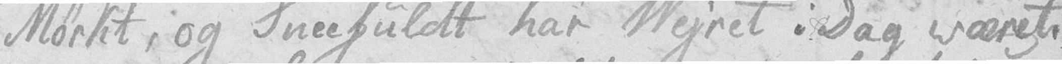Mørkt, og Sneefuldt har Vejret i Dag været.
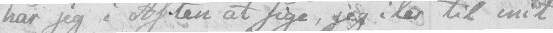har jeg i Aften at sige, jeg iler til mit
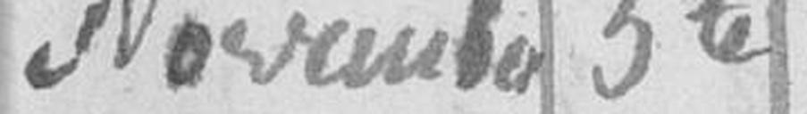November 5te
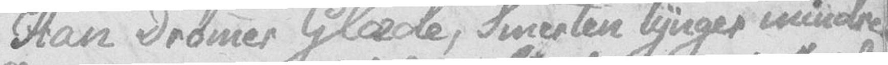Han Drømmer Glæde, Smerten tynger mindre.
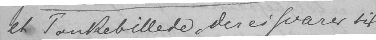et Tankebillede, der ei svarer til
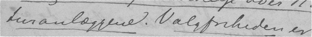turanlæggene. Valgfriheden er
